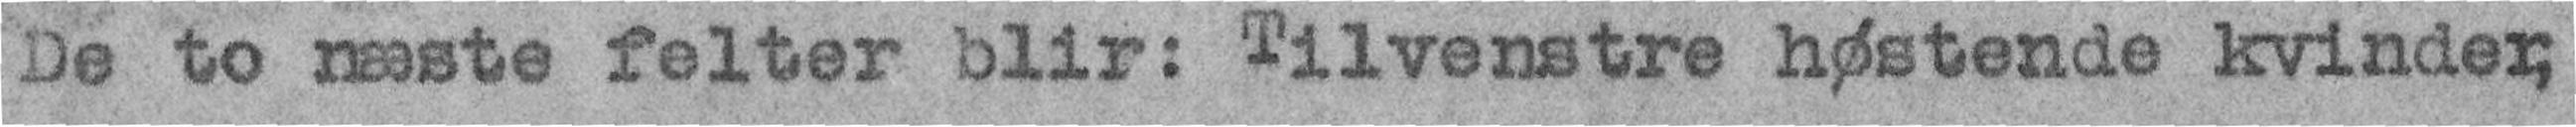De to næste felter blir: Tilvenstre høstende kvinder,
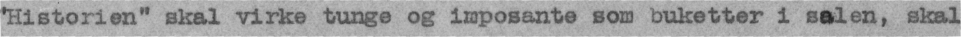«Historien» skal virke tunge og imposante som buketter i salen, skal
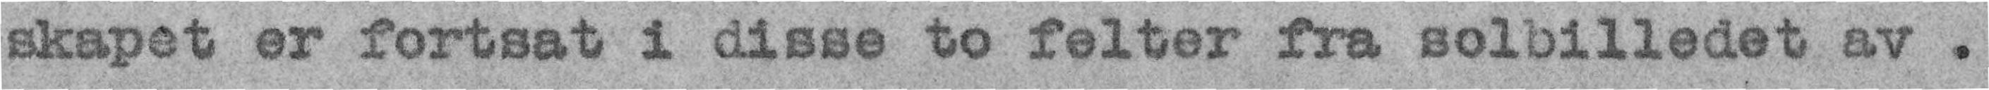skapet er fortsat i disse to felter fra solbilledet av.
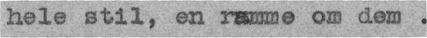hele stil, en ramme om dem.
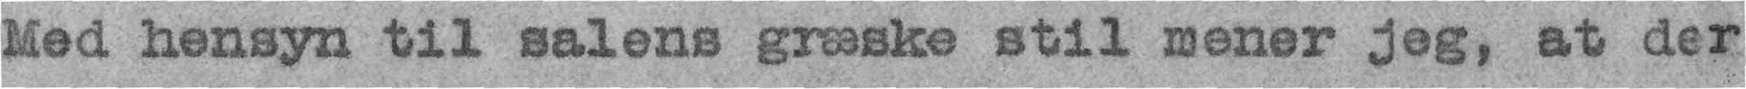Med hensyn til salens græske stil mener jeg, at der
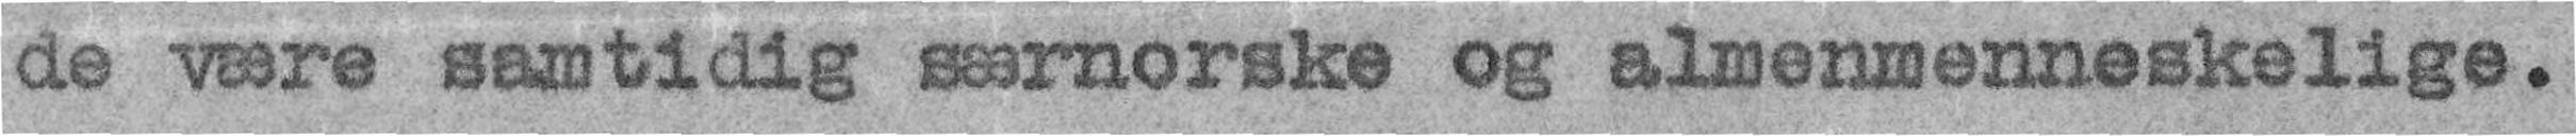de være samtidig særnorske og almenmenneskelige.
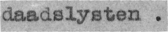daadslysten.
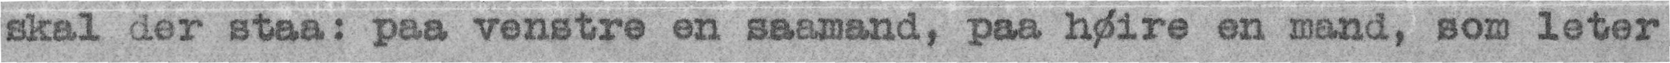skal der staa: paa venstre en saamand, paa høire en mand, som leter
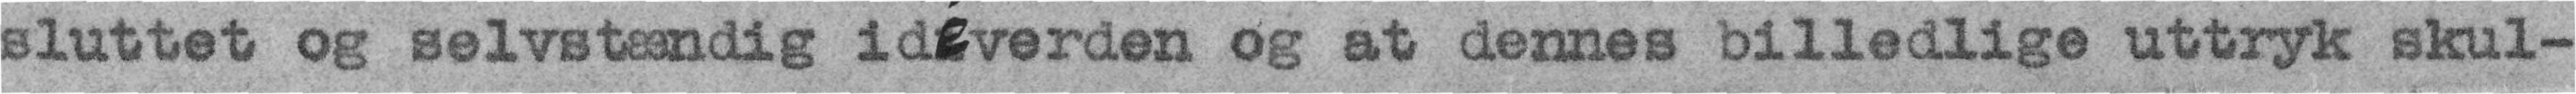sluttet og selvstændig idéverden og at dennes billedlige uttryk skul-
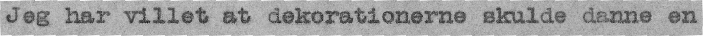Jeg har villet at dekorationerne skulde danne en
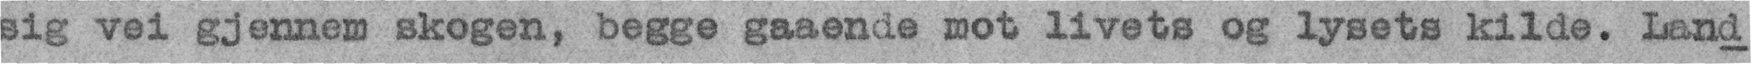sig vei gjennem skogen, begge gaaende mot livets og lysets kilde. Land-
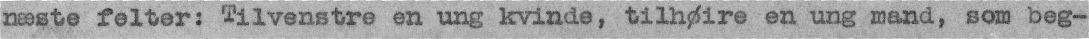næste felter: Tilvenstre en ung kvinde, tilhøire en ung mand, som beg-
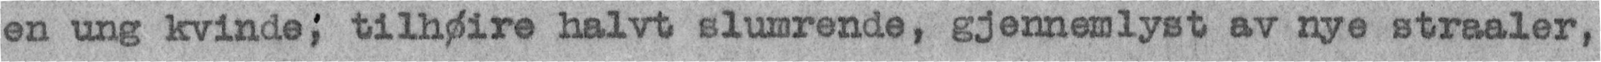en ung kvinde; tilhøire halvt slumrende, gjennemlyst av nye straaler,
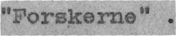«Forskerne».
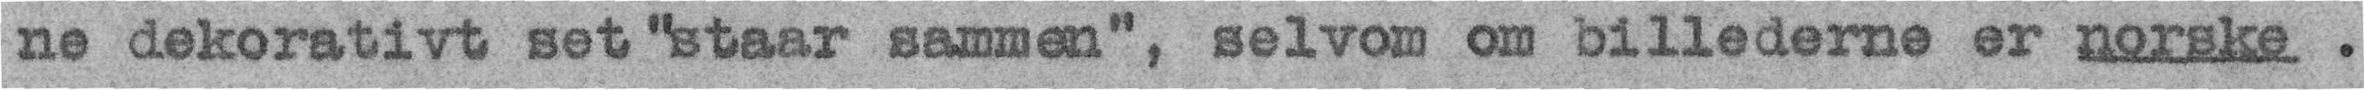ne dekorativt set «staar sammen», selvom om billederne er norske.
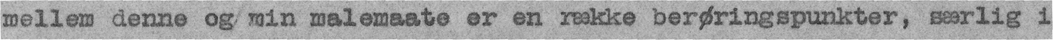mellem denne og min malemaate er en række berøringspunkter, særlig i
